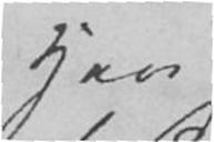Han
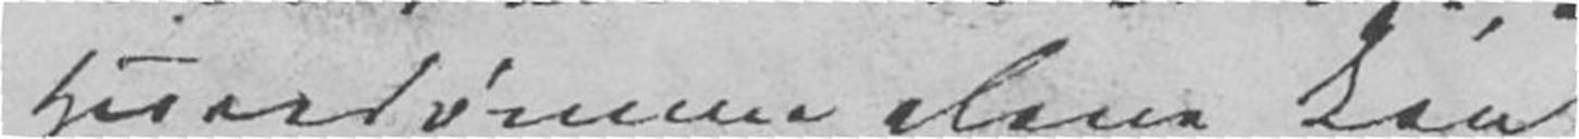Herredømme alene kan
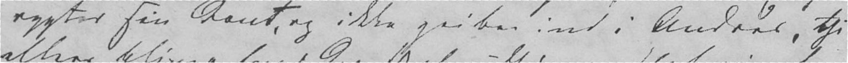rygter sin Dont, og ikke griber ind i Andres, thi
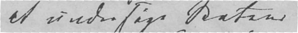at undersøge Statens
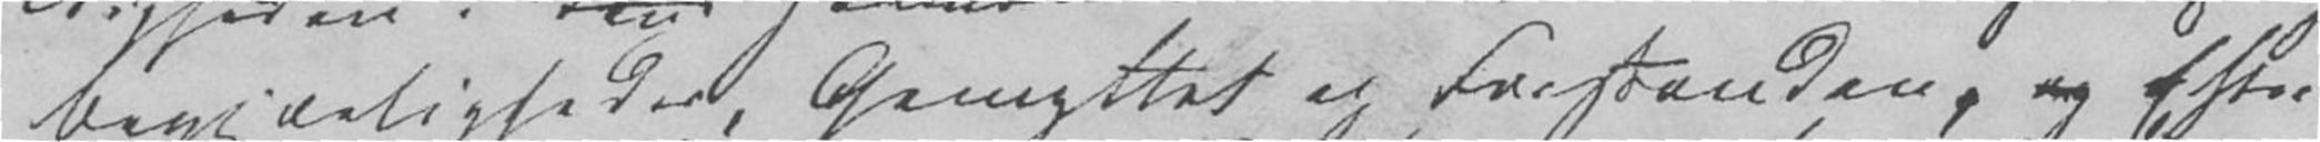Begjærligheder, Gemyttet og Forstanden., og Efter
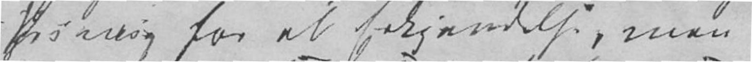Princip for al Erkjendelse, men
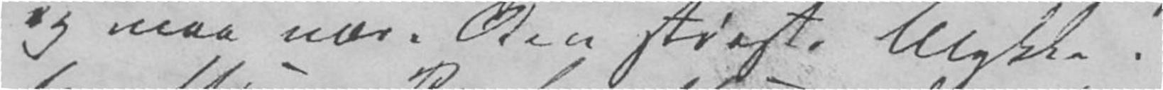og maa være den største Ulykke.
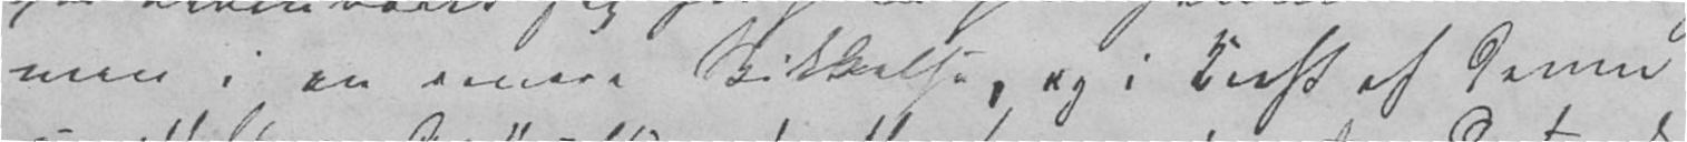men i en annen Skikkelse, og i Kraft af denne
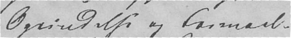Oprindelse og Formaal.
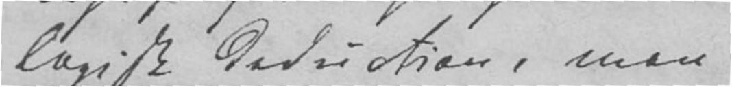logisk Deduction, men
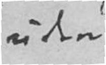uden
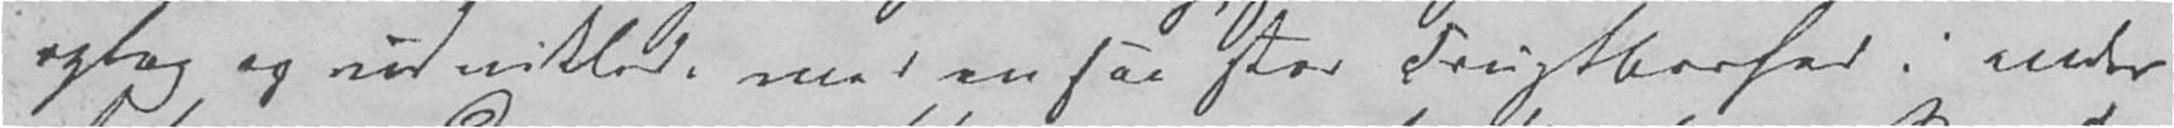optog og udviklede med en saa stor Frugtbarhed i anden
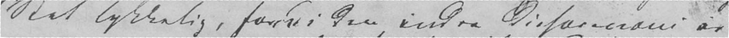Stat lykkelig, fordi den indre Disharmoni er
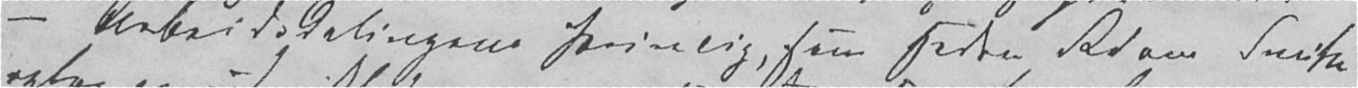– Arbeidsdelingens Princip, som siden Adam Smith
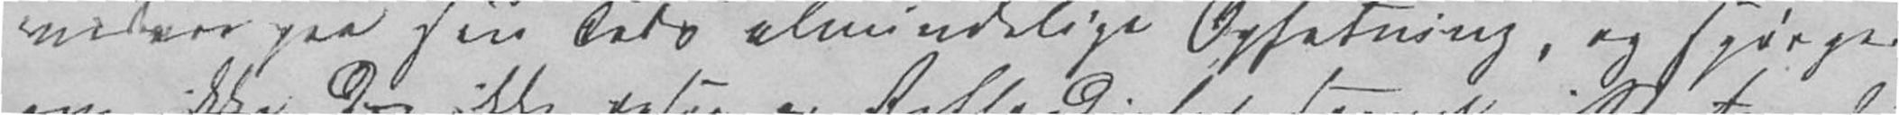videre paa sin Tids almindelige Opfatning, og spørger
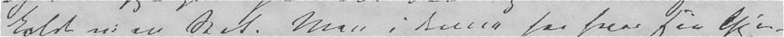kalde vi en Stat. Men i denne har hver sin Gjer-
